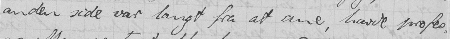anden side var langt fra at ane, havde profes-
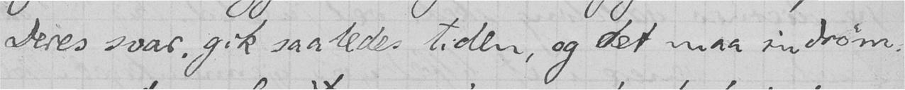Deres svar, gik saaledes tiden, og det maa indrøm-
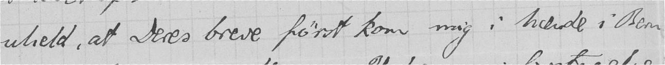uheld, at Deres breve først kom mig i hænde i Bern
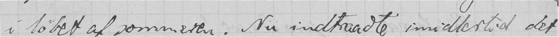i løbet af sommeren. Nu indtraadte imidlertid det
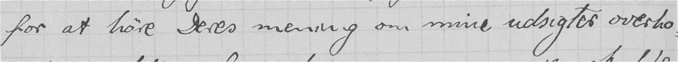for at høre Deres mening om mine udsigter overho-
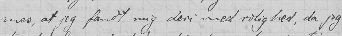mes, at jeg fandt mig deri med rolighed, da jeg
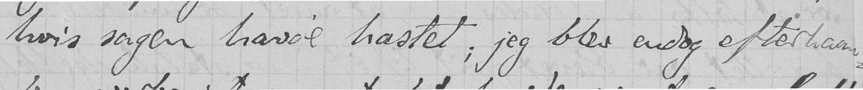hvis sagen havde hastet; jeg blev endog efterhaan-
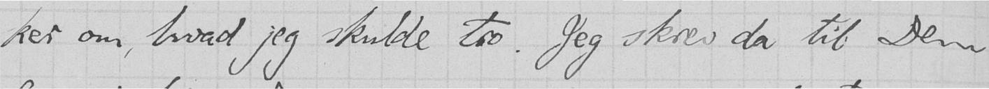ker om, hvad jeg skulde tro. Jeg skrev da til Dem
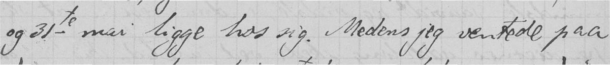og 31te mai ligge hos sig. Medens jeg ventede paa
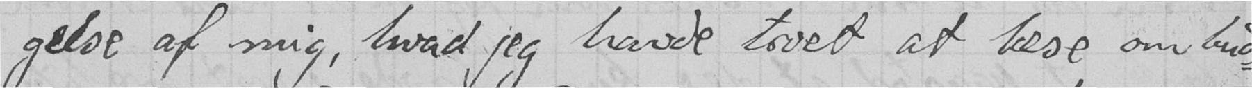gelse af mig, hvad jeg havde troet at læse om bud-
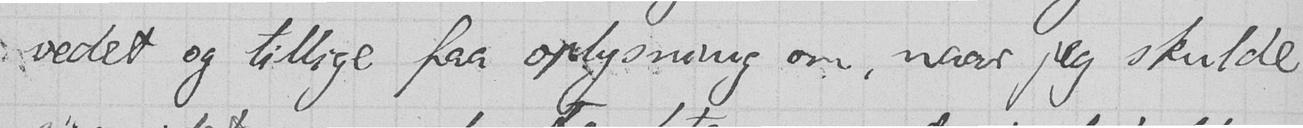vedet og tillige faa oplysning om, naar jeg skulde
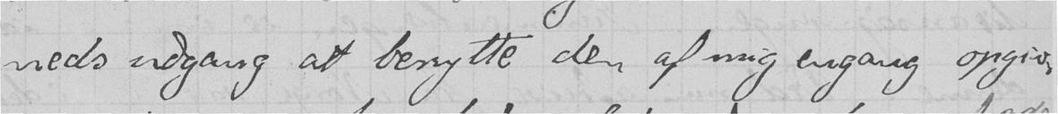neds udgang at benytte den af mig engang opgiv-
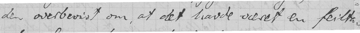den overbevist om, at det havde været en feilta-
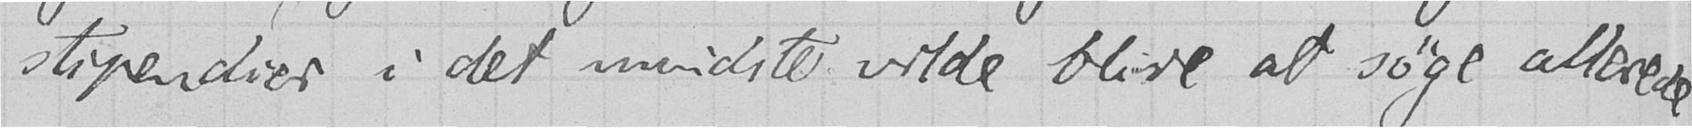stipendier i det mindste vilde blive at søge allerede
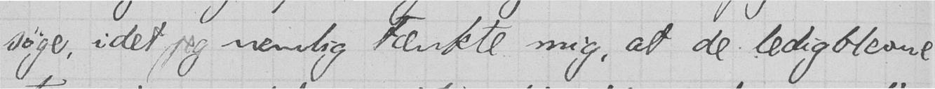søge, idet jeg nemlig tænkte mig, at de ledigblevne
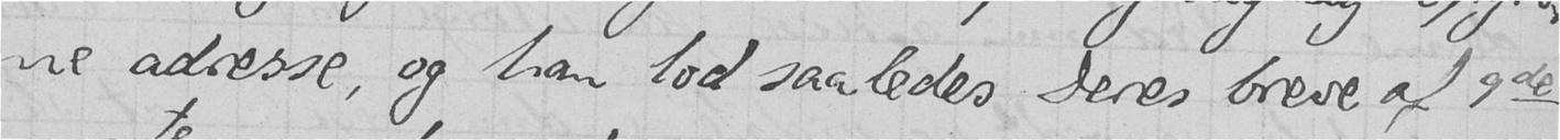ne adresse, og han lod saaledes Deres breve af 9de
